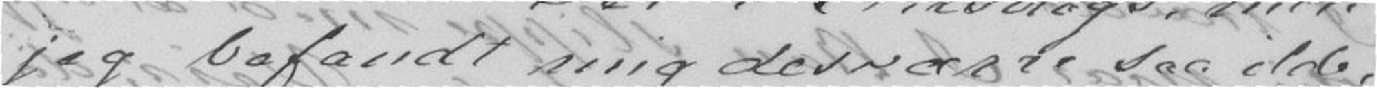jeg befandt mig desværre saa ilde;
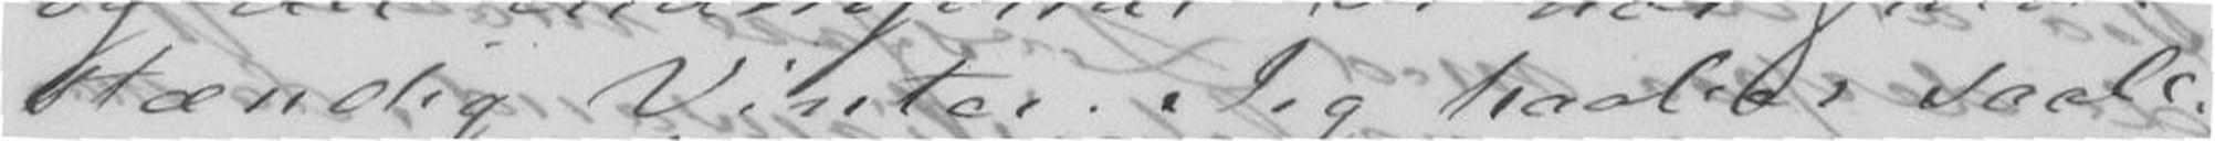stændig Vinter. Jeg haaber saale-
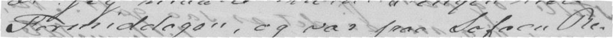Formiddagen, og var paa Sofaen Re-
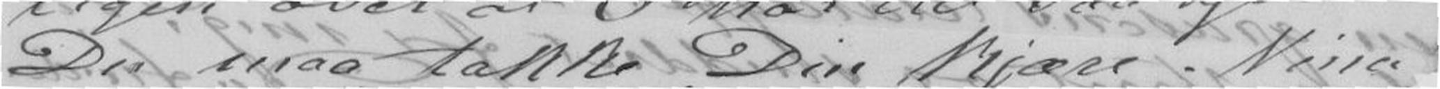Du maa takke Din kjære Nina
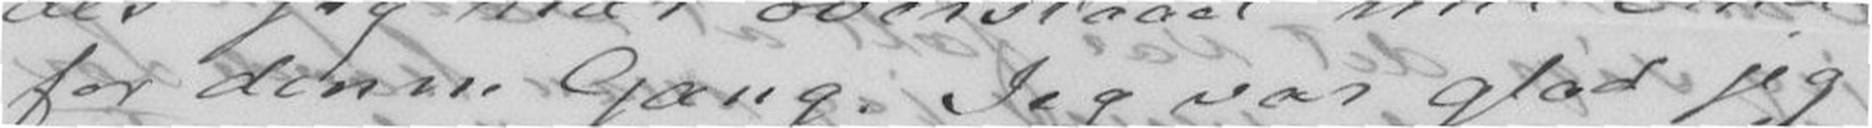for denne Gang. Jeg var glad jeg
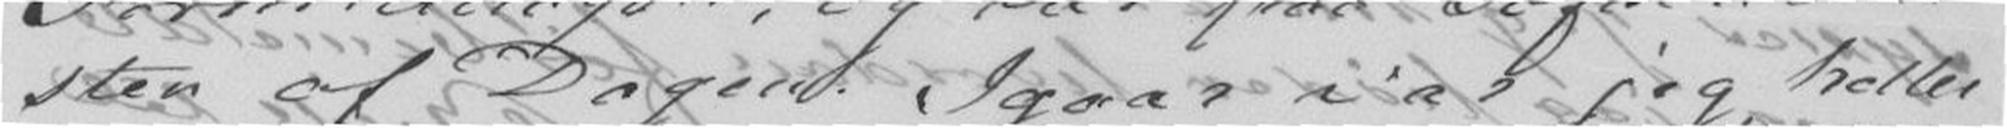sten af Dagen. Igaar var jeg heller
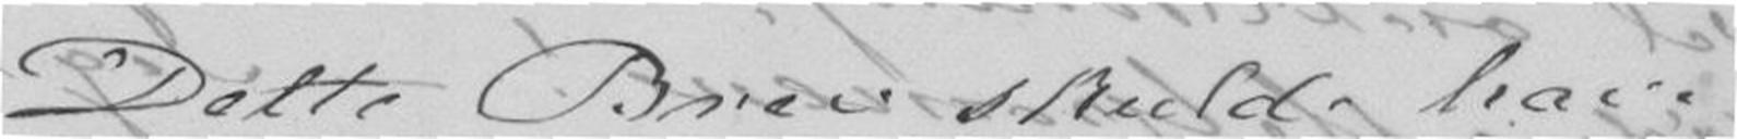Dette brev skulde have
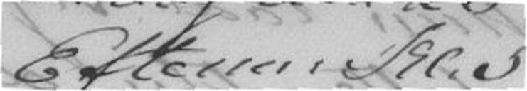Efterm. Kl.5
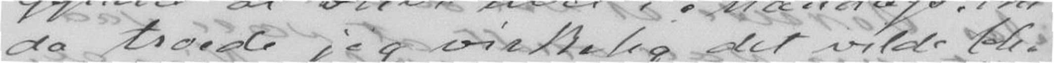da troede jeg virkelig det vilde bli-
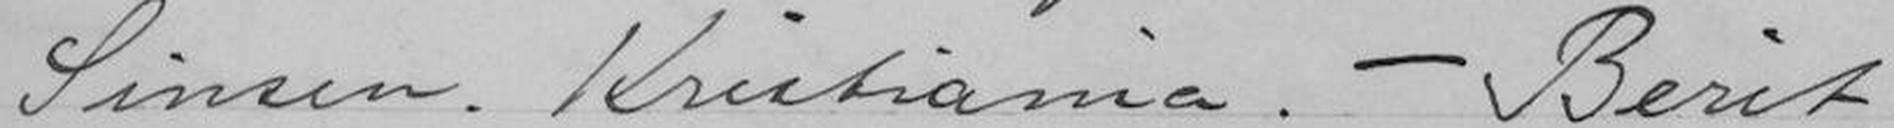Sinsen. Kristiania. - Berit,
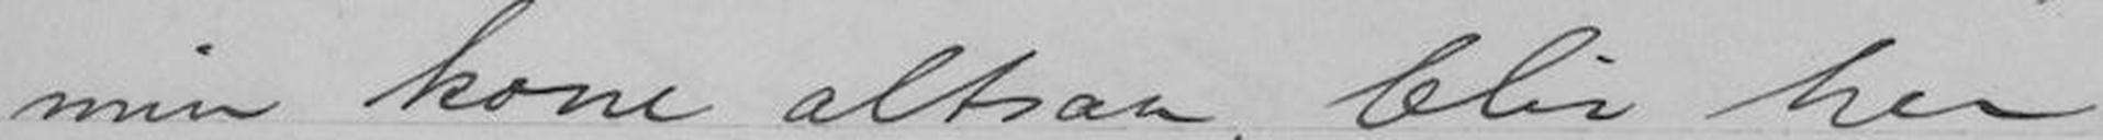min kone altsaa, blir her
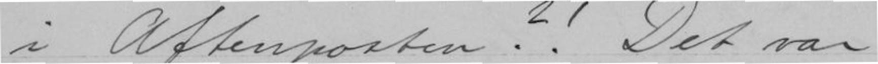i Aftenposten?! Det var
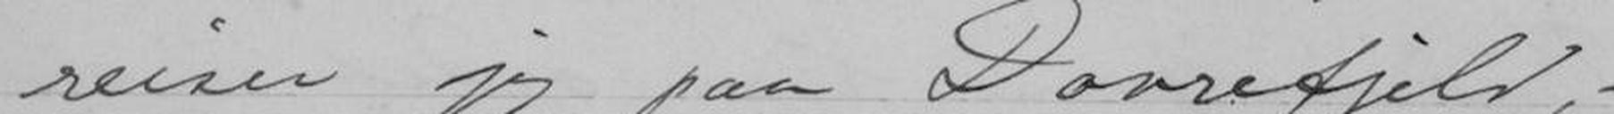reiser jeg paa Dovrefjeld, -
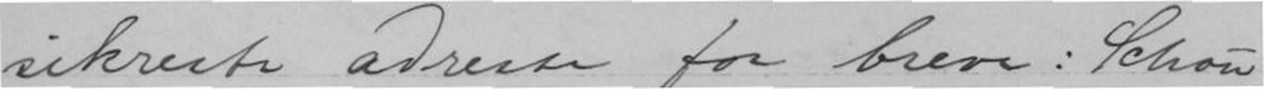sikreste adresse for breve: Schou,
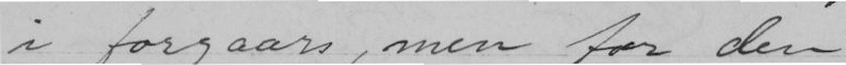i forgaars, men for den
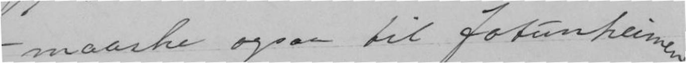- maaske ogsaa til Jotunheimen,
